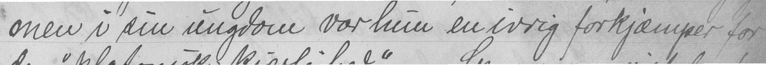men i sin ungdom var hun en ivrig forkjæmper for
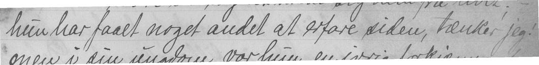hun har faaet noget andet at erfare siden, tænker jeg!
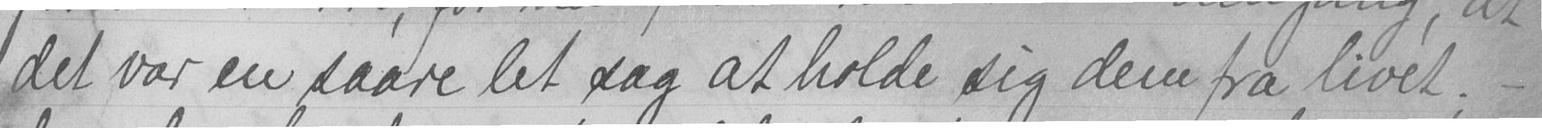det var en saare let sag at holde sig dem fra livet; -
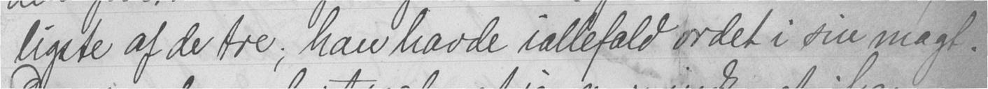ligste af de tre; han havde iallefald ordet i sin magt.
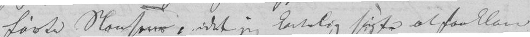førte Slaasom, idet jeg endelig søgte at forklare
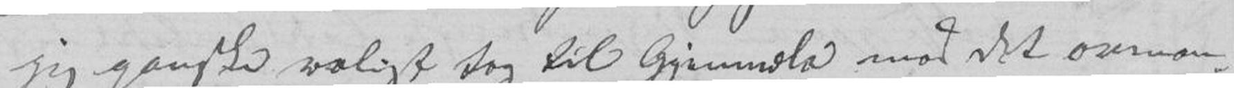jeg ganske roligt tog til Gjenmæle mod det ovenom-
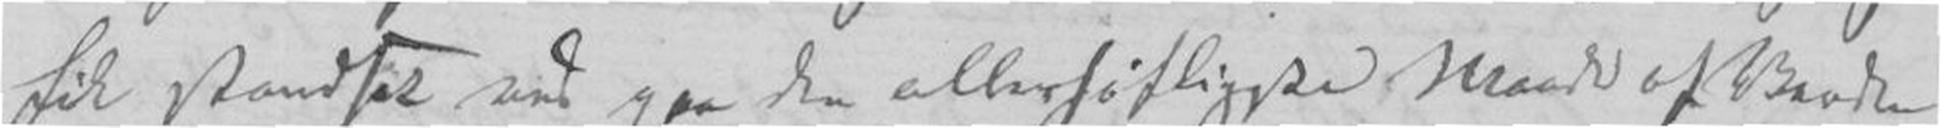fik standset end paa den allerhøfligste Maade af Baade
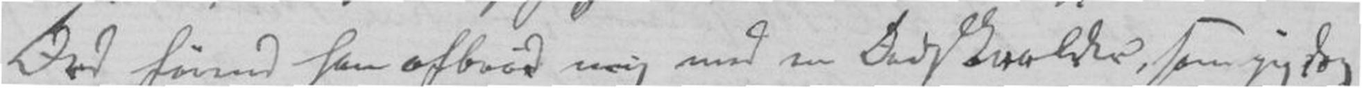Ord førend han afbrød mig med en Ordskvalder, som jeg dog
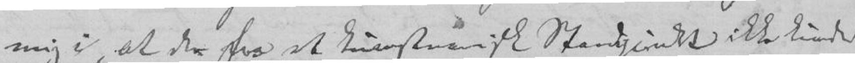mig i, at der fra et kunstnærisk Standpunkt ikke kunde
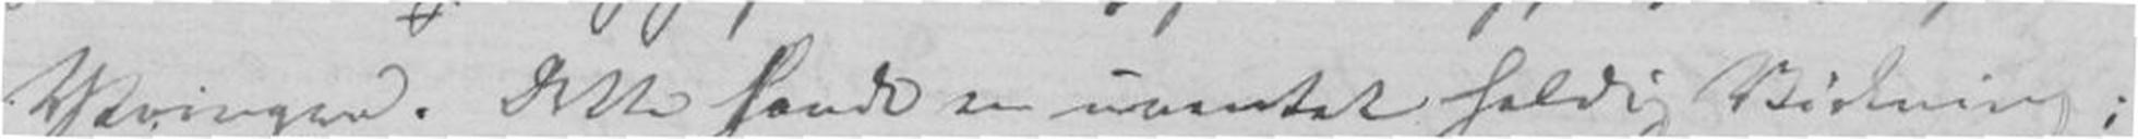Ytringer. Dette havde en uventet heldig Virkning;
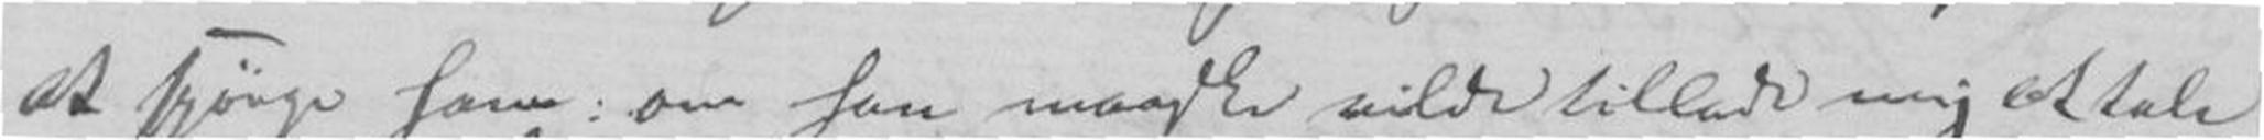at spørge ham: om han maaske vilde tillade mig at tale
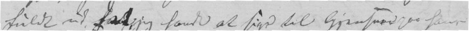fuldt ud jeg havde at sige til Gjensvar paa hans
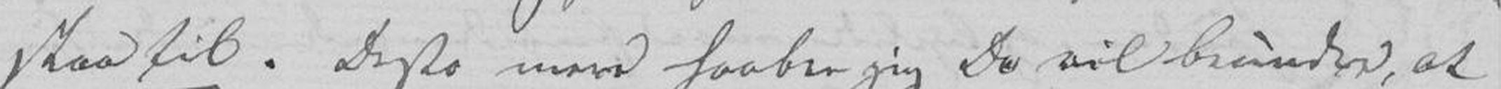at staa til. Desto mer haaber jeg De vil beundre, at
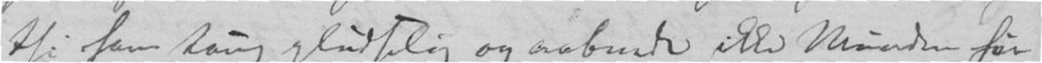thi han taug pludselig og aabenede ikke munden før
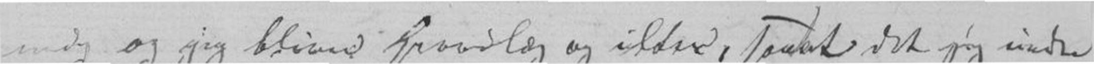mig og jeg bliver G..læg og ildter, saa at det jeg indre
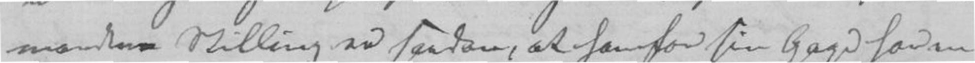manden Stilling som saadan, at han for sin Gage saadan
